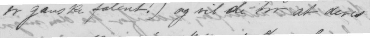er ganske fælent!) og vil de tro at deres
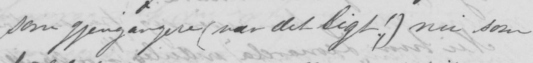som gjengangere (var det ligt!,) nei som
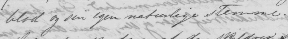blod og sin egen naturlige stemme.
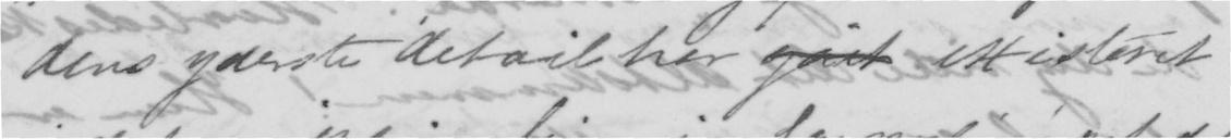deres yderste detail har existeret
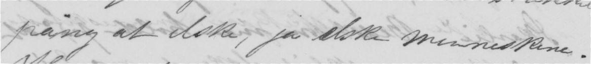påny at elske, ja elske menneskene.
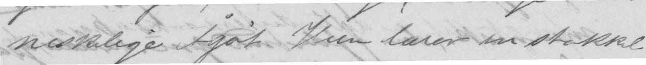neskelige Ågåt. Hun lærer en stakkel
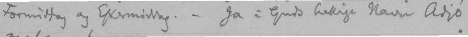Formiddag og Eftermiddag. - Ja i Guds hellige Navn Adjø
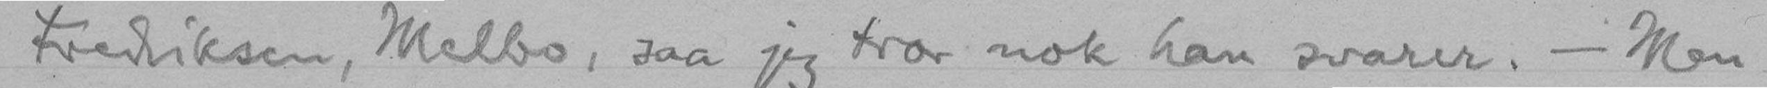Fredriksen, Melbo, saa jeg tror nok han svarer. - Men
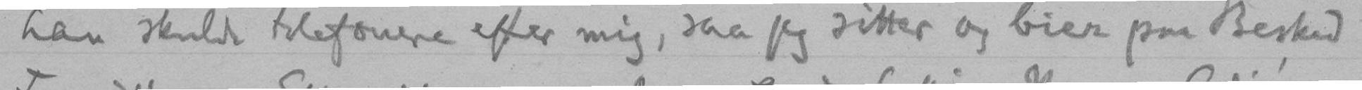han skulde telefonere efter mig, saa jeg sitter og bier paa Besked
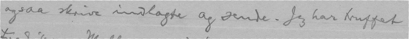ogsaa skrive indlagte og sende. Jeg har truffet
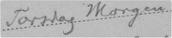Torsdag Morgen
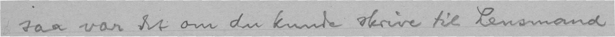saa var det om du kunde skrive til Lensmand
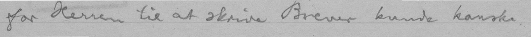for Herren til at skrive Brever kunde kanske
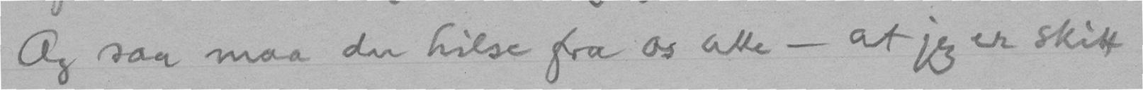Og saa maa du hilse fra os alle - at jeg er Skitt
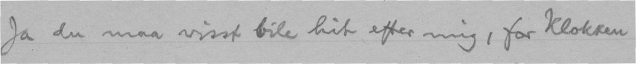Ja du maa visst bile hit efter mig, for Klokken
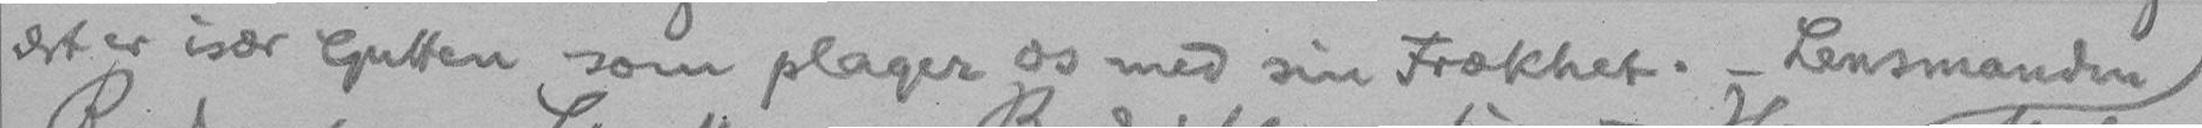det er især Gutten som plager os med sin Frækhet. - Lensmanden
